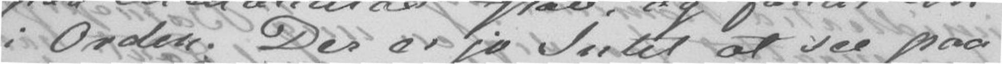i Orden. Der er jo Intet at see paa
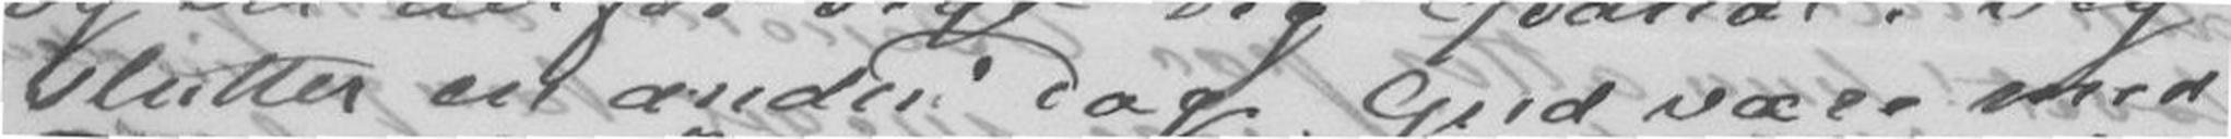slutter en anden Dag Gud være med
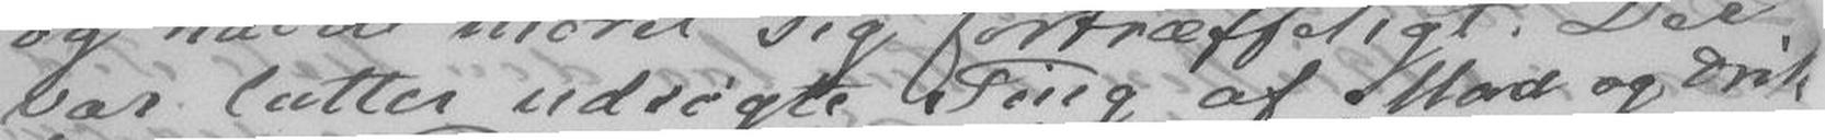var lutter udsøgte Ting af Mad og Drik-
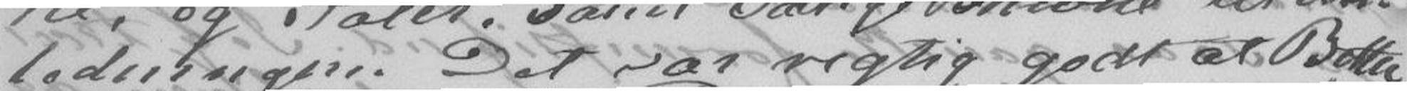ledningen. Det var rigtig godt at Betten
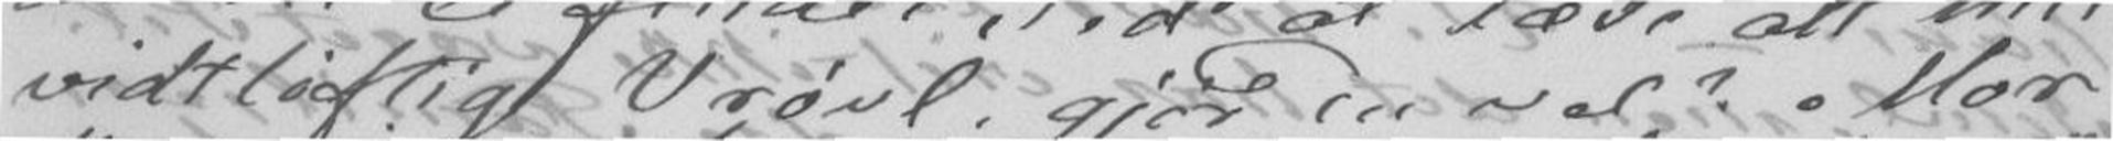vidtløftig Vrøvl, gjør Du vel? Mor
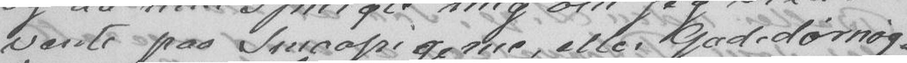vænte paa Smaapigerne, eller Gadedørnøg-
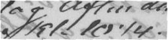Kl. 10¼.
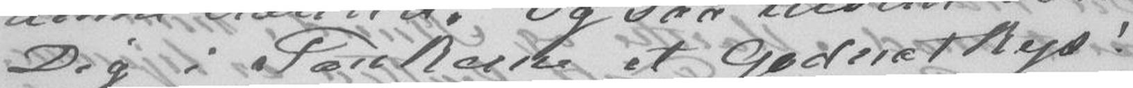Dig i Tankerne et godnatkys!
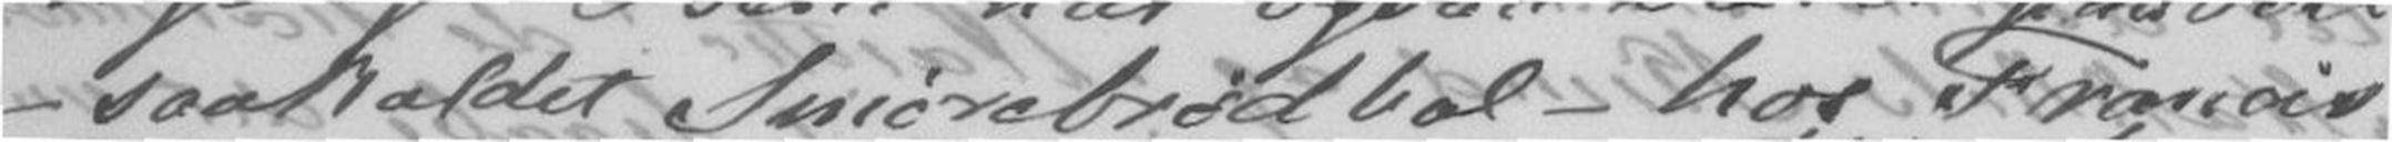- saakaldet Smørebrødbal - hos Francis
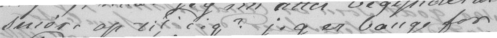smøre op til Dig? jeg er bange for
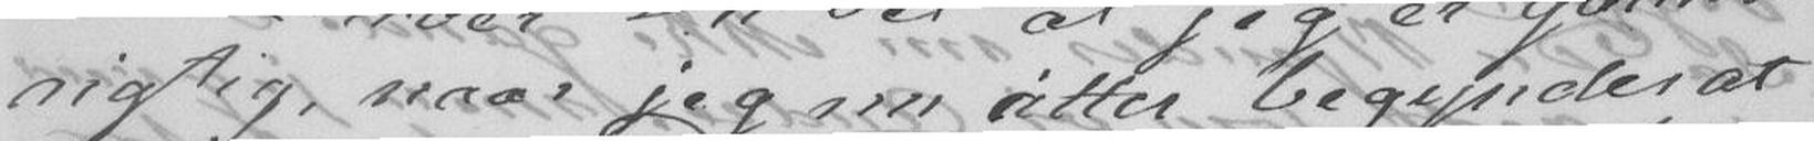rigtig, naar jeg nu àtter begynder at
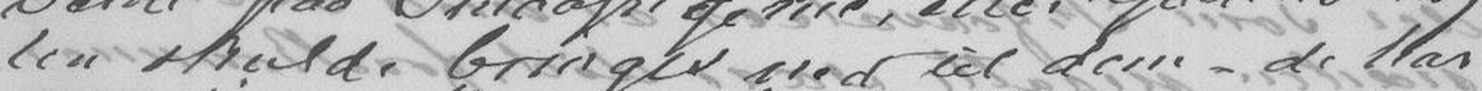len skulde bringes ned til dem - de har
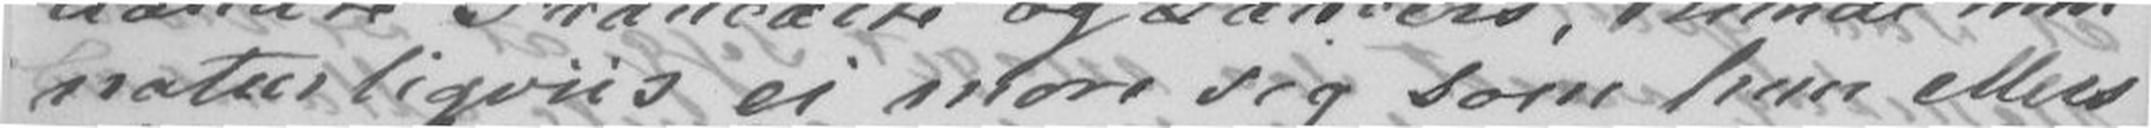naturligviis ei more sig som hun ellers
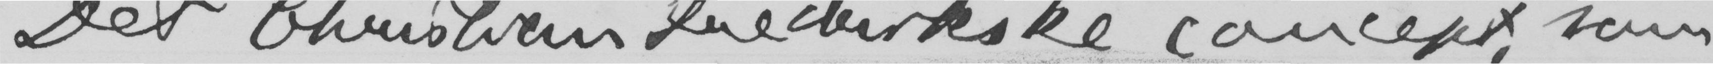Det Christian Fredrikske concept, som
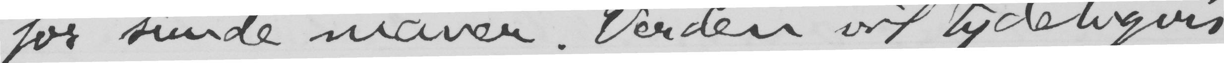for sunde maver. Verden vil tydeligvis
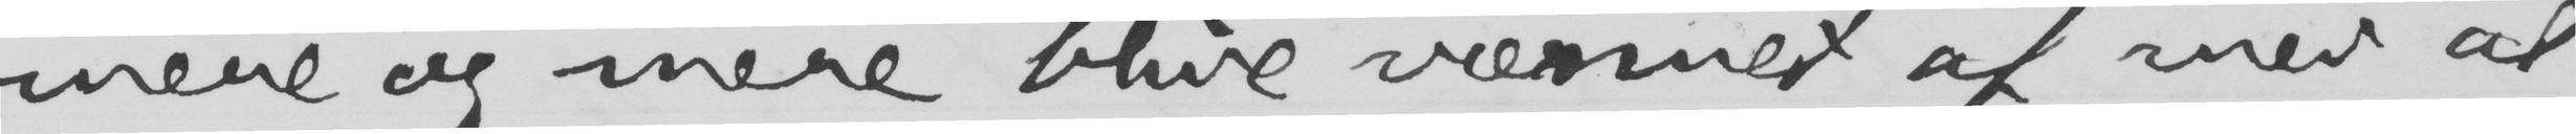mere og mere blive vænnet af med at
